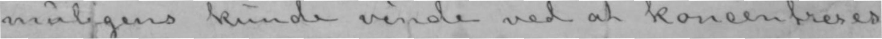muligens kunde vinde ved at koncentreres;
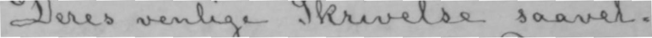Deres venlige Skrivelse saavel-
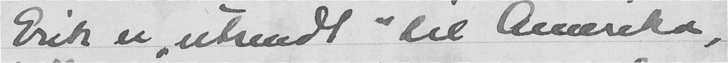Erik er "utsendt" til Amerika,
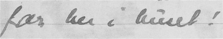far her i huset!
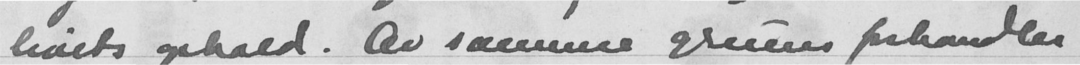livets ophold. Av samme grunn forhandler
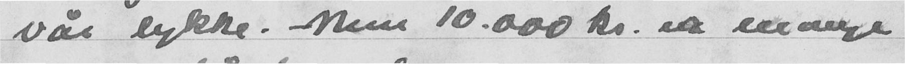vår lykke. Men 10.000 kr. er mange
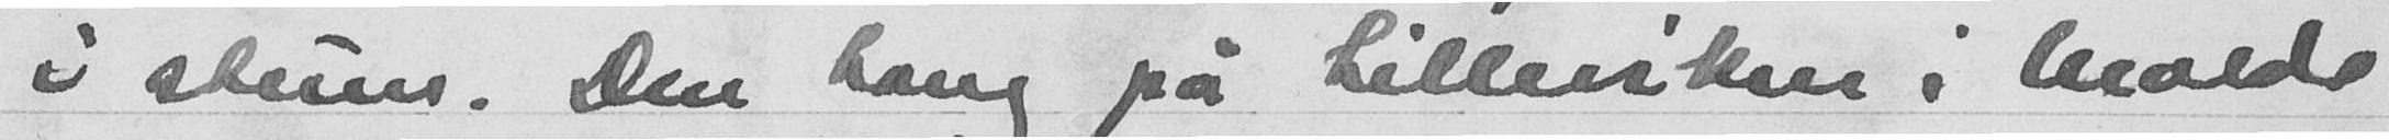i stuen. Den hang på lilleviken i Molde
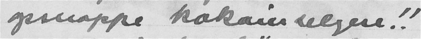opsnappe kokainiselgere.
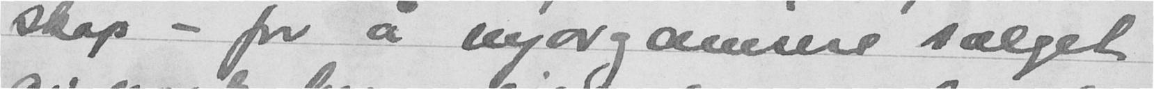skap - for å nyorganisere salget
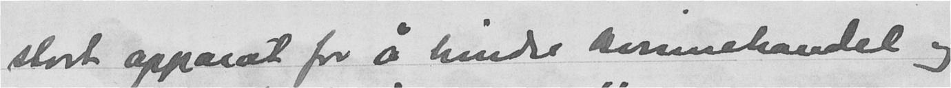stort apparat for å hindre kvinnehandel og
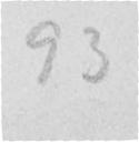93
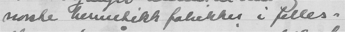norske Hermetikkfabrikker i felles-
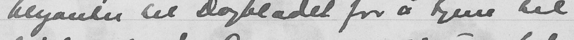blyanter til Dagbladet for å tjene til
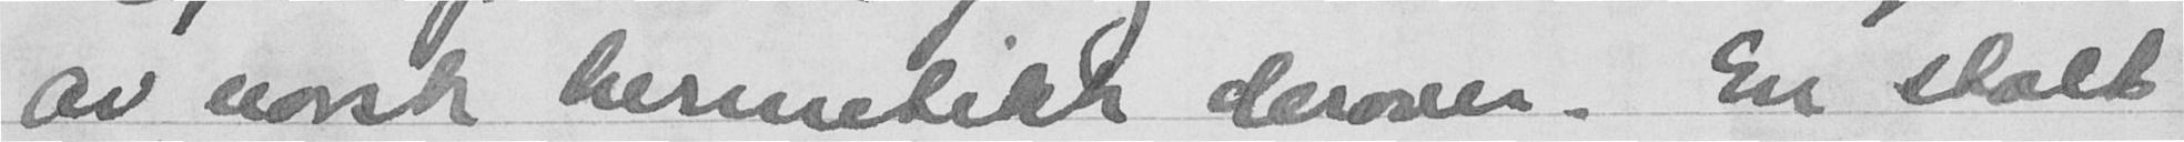av norsk hermetikk derover. En stolt
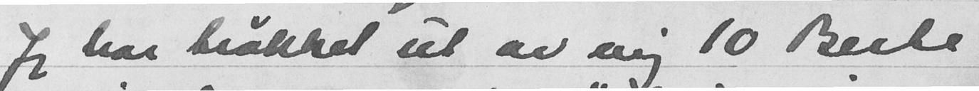Jeg har trøkket ut av mig 10 Beste
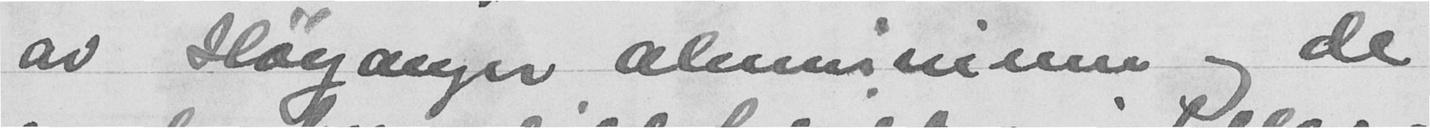av Høyanger aliminium og de
